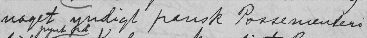noget yndigt fransk Possementeri
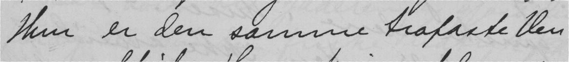Hun er den samme trofaste ven
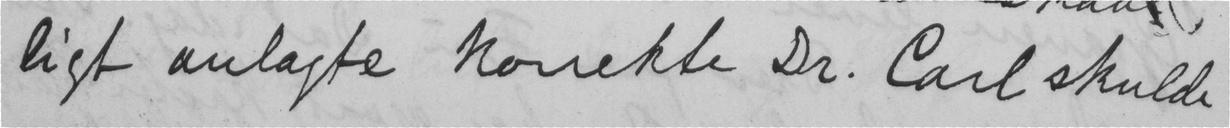ligt anlagte korrekte Dr. Carl skulde
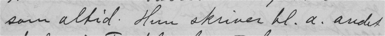som altid. Hun skriver bl.a. andet
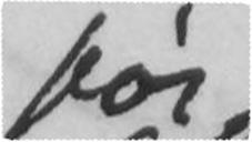før
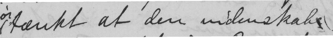tænkt at den videnskab-
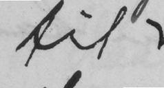til
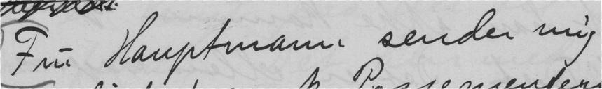Fru Hauptmann sender mig
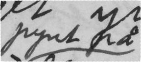pynt på
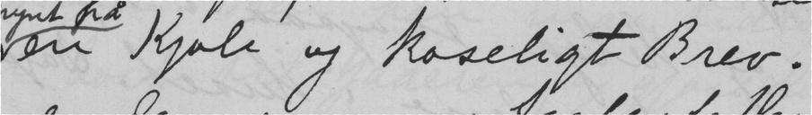en Kjole og koseligt Brev.
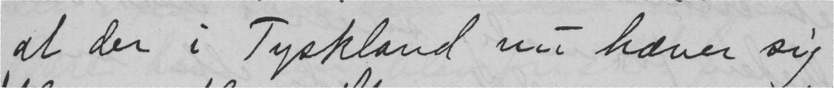at der i Tyskland nu hæver sig
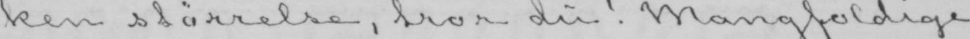ken størrelse, tror du! Mangfoldige
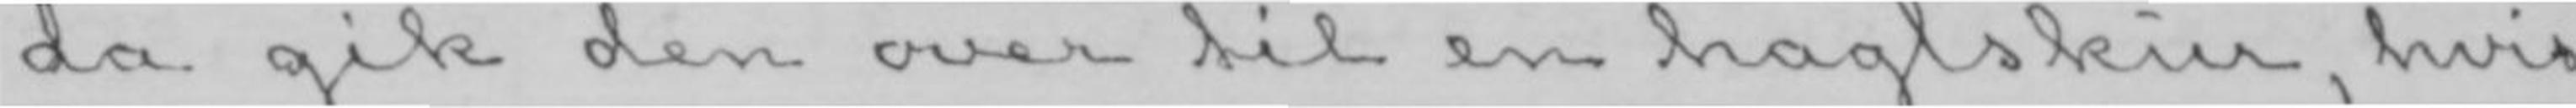da gik den over til en haglskur, hvis
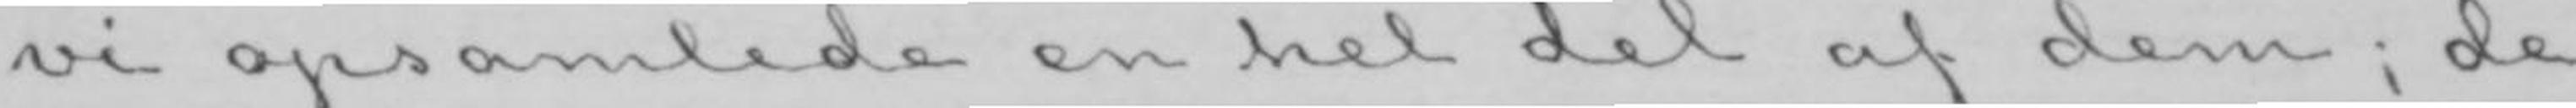vi opsamlede en hel del af dem; de
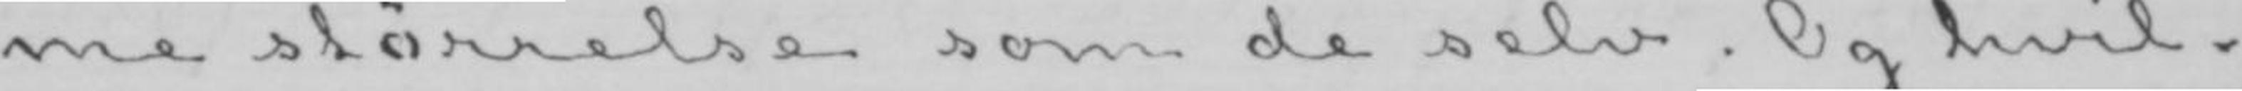me størrelse som de selv. Og hvil-
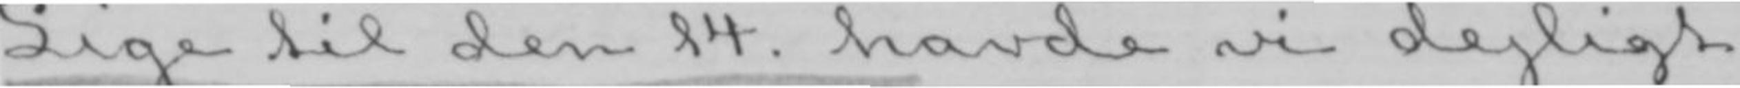Lige til den 14. havde vi dejligt
